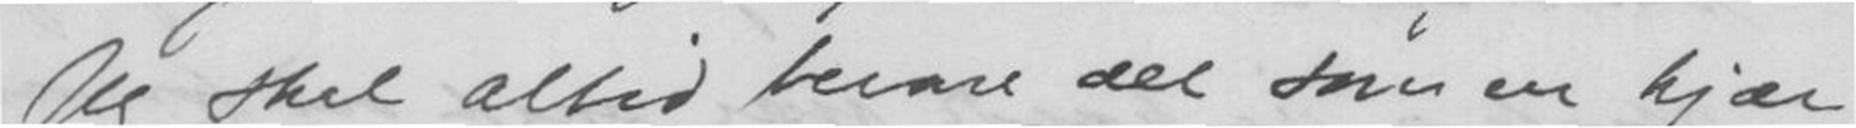Jeg skal altid bevare det som en kjær
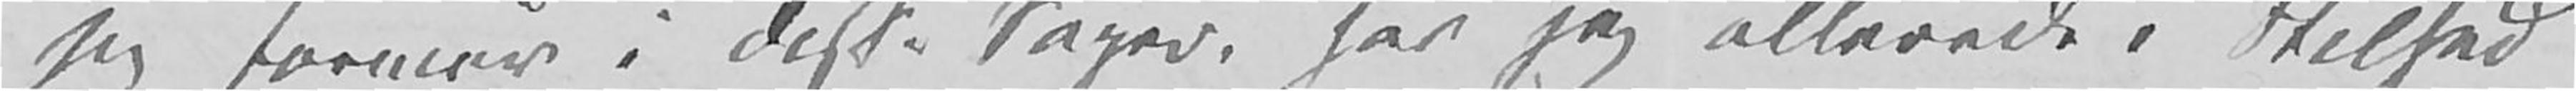jeg formår i disse Sager, har jeg allerede i Stilhed
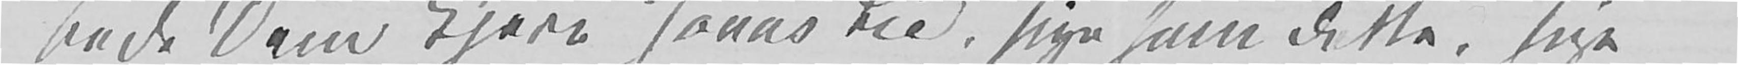bede Dem kjere Jonas Lie, sige ham dette, sige
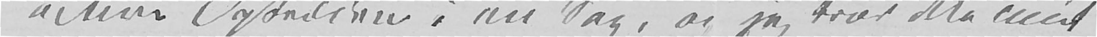active Optræden i en Sag, og jeg tror ikke mit
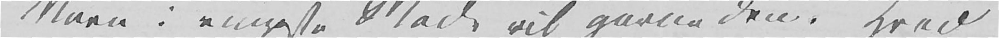Navn i ringeste Måde vil gavne den. Hvad
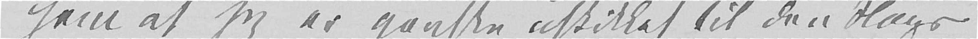ham at jeg er ganske uskikket til den Slags
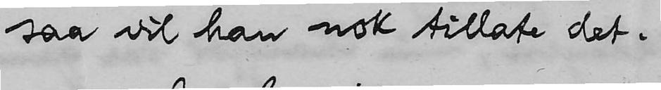saa vil han nok tillate det.
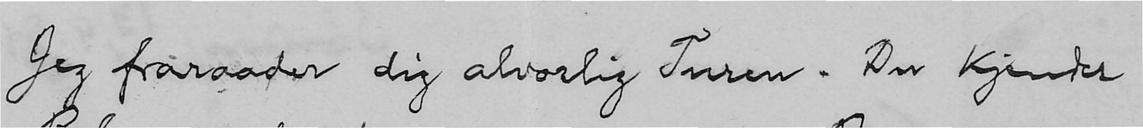Jeg fraraader dig alvorlig Turen. Du kjender
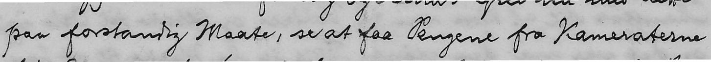paa forstandig Maate, se at faa Pengene fra Kameraterne
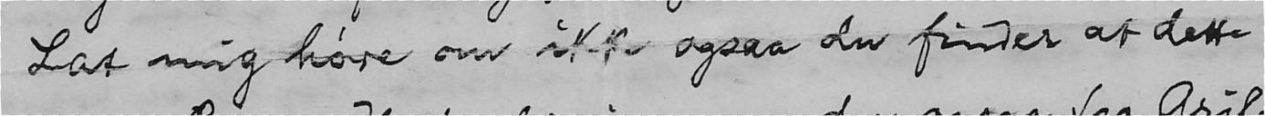Lat mig høre om ikke ogsaa du finder at dette
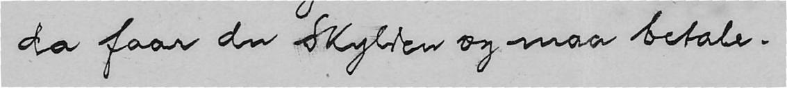da faar du Skylden og maa betale.
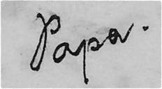Papa.
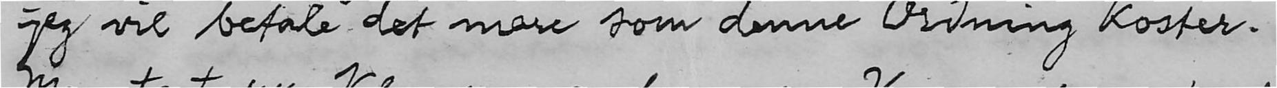jeg vil betale det mere som denne Ordning koster.
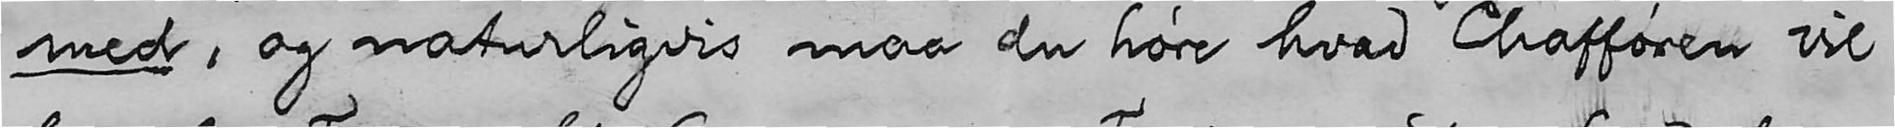med, og naturligvis maa du høre hvad Chafføren vil
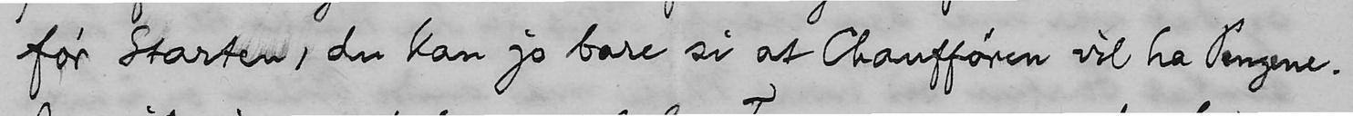før Starten, du kan jo bare si at Chaufføren vil ha Pengene.
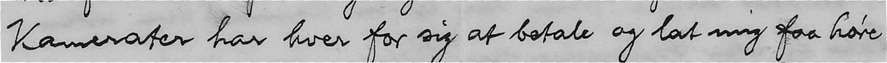Kamerater har hver for sig at betale og lat mig faa høre
